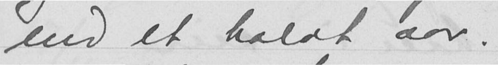end et halvt aar.
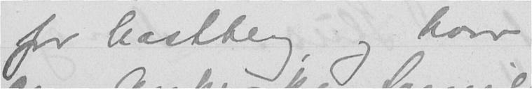for Castberg, og hvor
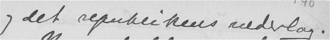og det republikens nederlag.
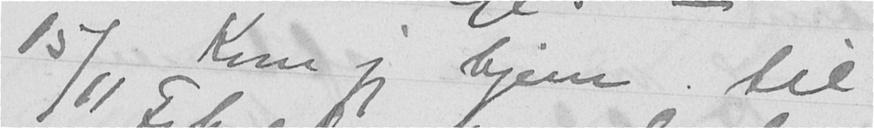15/11 Kom jeg hjem til
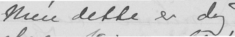Men dette er dog
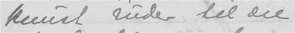knust ruder til ære
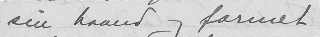sin haand og formet
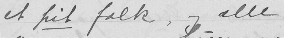et frit folk, og alle
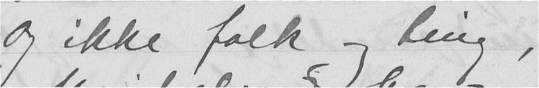Og ikke folk og ting,
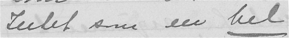Intet som en hel
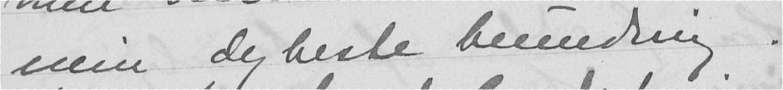men dybeste beundring.
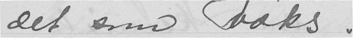det som voks.
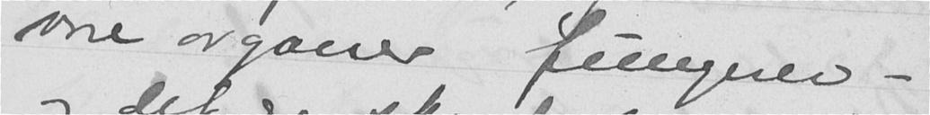vore organer fungerer -
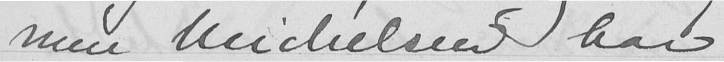men Michelsen har
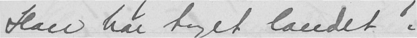Han har taget landet i
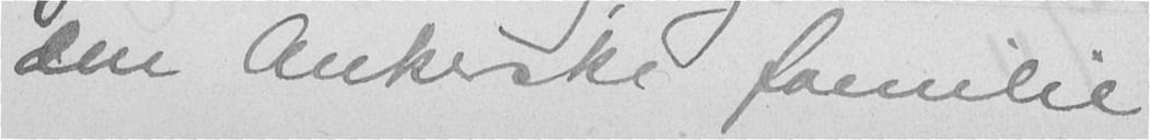den Ankerske familie
